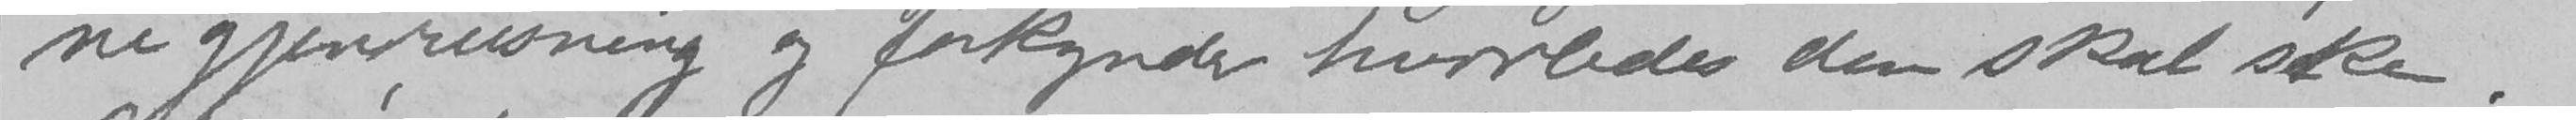ne gjenreisning og forkynder hvorledes den skal ske.
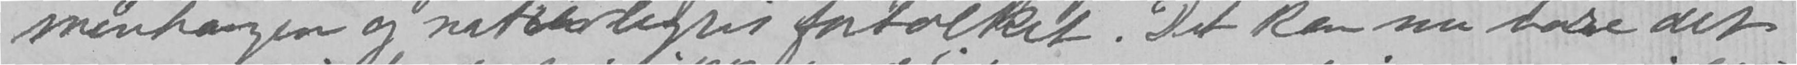menhængen og naturligvis fortolket. Det kan nu være det
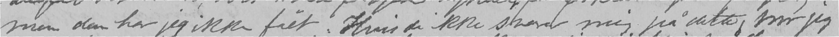men den har jeg ikke fået. Hvis de ikke svarer mig på dette, tror jeg
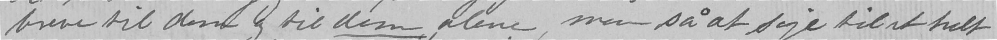breve til dem og til dem alene, men så at sige til et helt
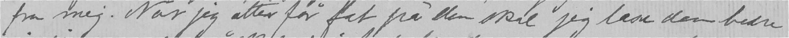fra mig. Når jeg atter får fat på den skal jeg læse den bedre
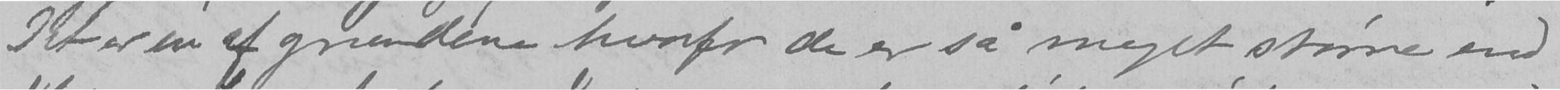Det er en af grundene hvorfor de er så meget større end
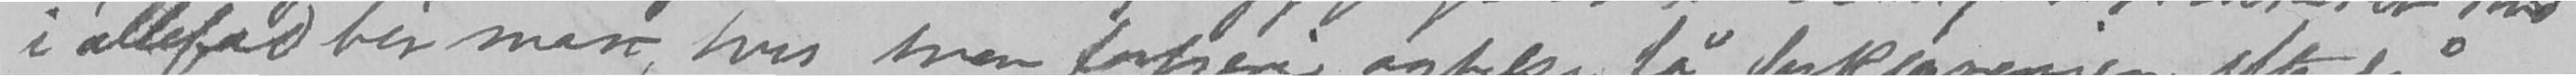i allefald bør man, hvis man fortjener agtelse, få forklaringen efterpå,
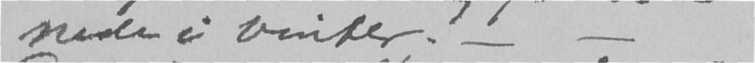nede i vinter. - -
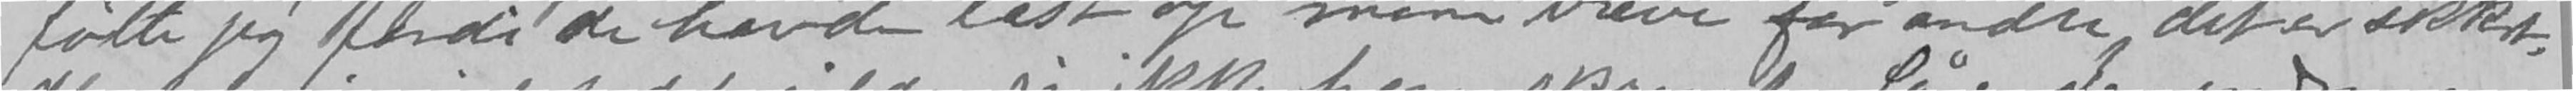følte jeg fordi de havde lest op mine breve for andre, det er sikkert.
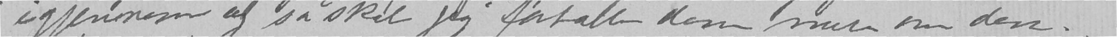igjennem og så skal jeg fortælle dem mere om den.
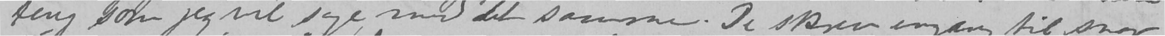ting som jeg vil sige med det samme. De skrev engang til svar
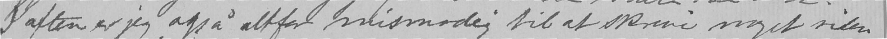Iaften er jeg også altfor mismodig til at skrive noget såden
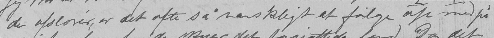de afslører, er det ofte så vanskligt at følge med på
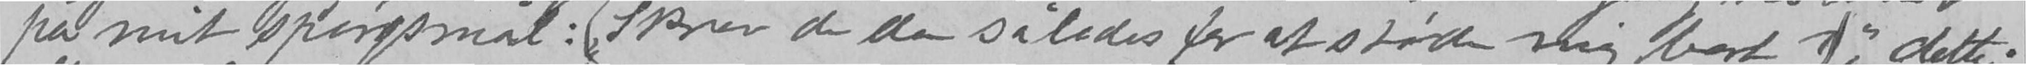på mit spørgsmål: ("Skrev de da således for at støde mig bort?)", dette:
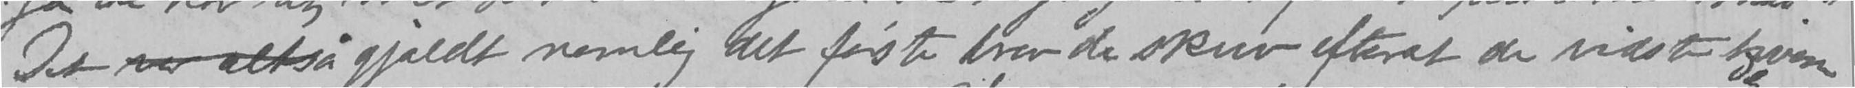Det var altså gjaldt nemlig det første brev de skrev efterat de vidste hvem
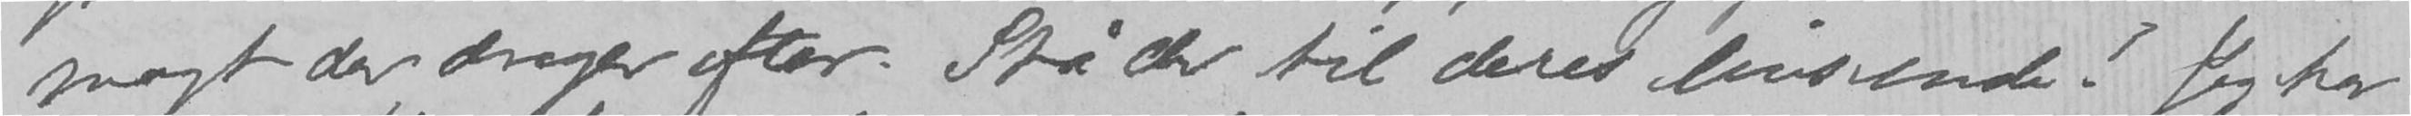magt der drager efter. Stå der til deres livs ende! Jeg har
